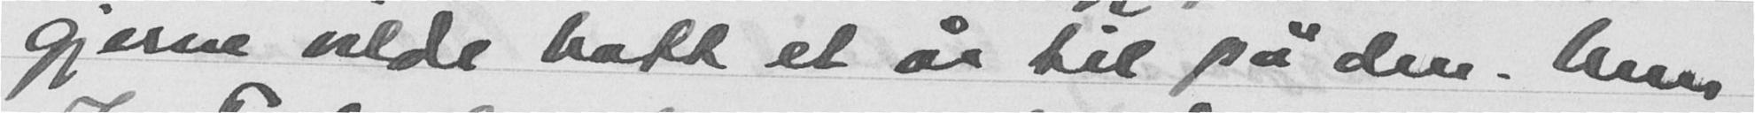gjerne vilde hatt et år til på den. Men
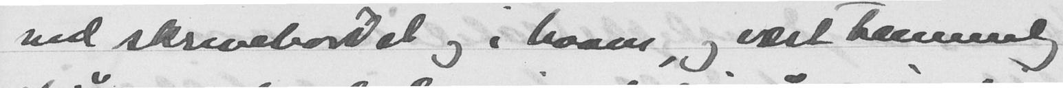ved skrivebordet og i haven, og vært temmelig
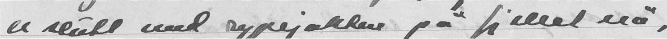er slutt med rypejakten på fjellet nå,
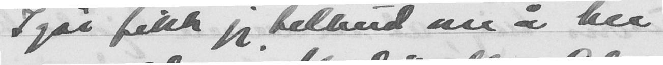Igår fikk jeg tilbud om å bli
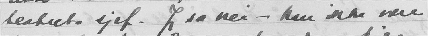teatrets sjef. Jeg sa nei - kan ikke være
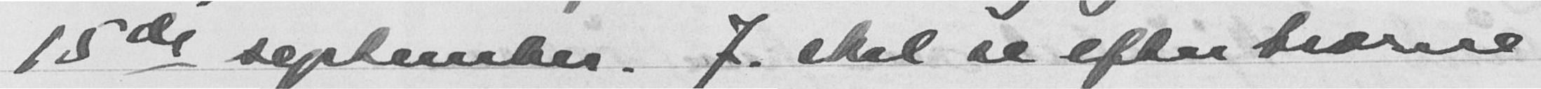15de september. J. skal se efter trærne
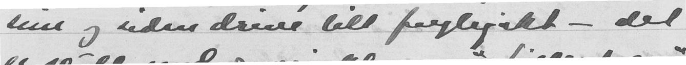sine og siden drive litt fuglejakt - det
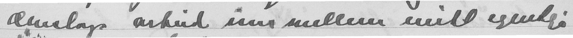denslags arbeid inniemellom mitt egentlige.
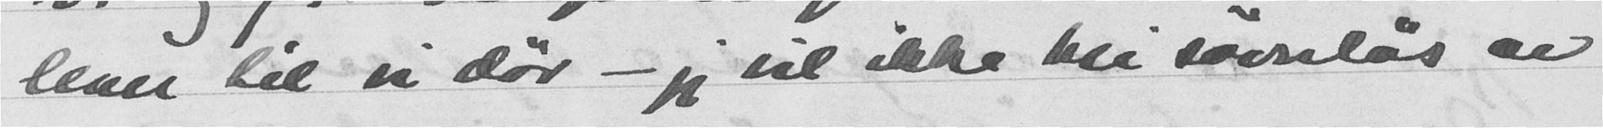lever til vi dør - jeg vil ikke bli søvnløs av
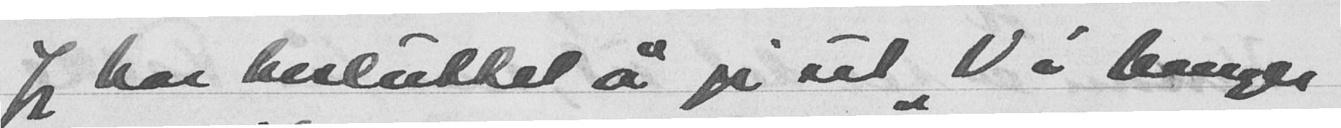Jeg har besluttet å gi ut "Vi henger
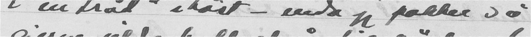i en tråd" i høst - enda jeg pokker så
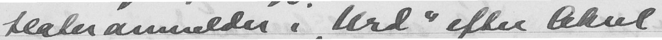teateranmelder i "Vrd" efter Aksel
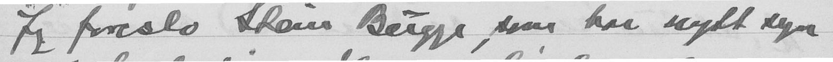Jeg foreslo Stein Bugge, som har nytt tegn
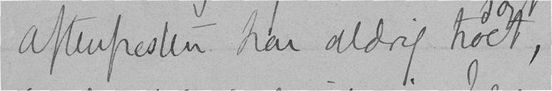Aftenposten har aldrig troet,
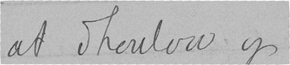at Thaulow og
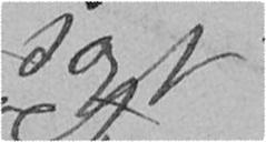sagt
